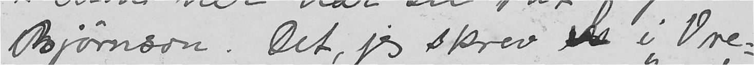Bjørnson. Det, jeg skrev i "Vre-
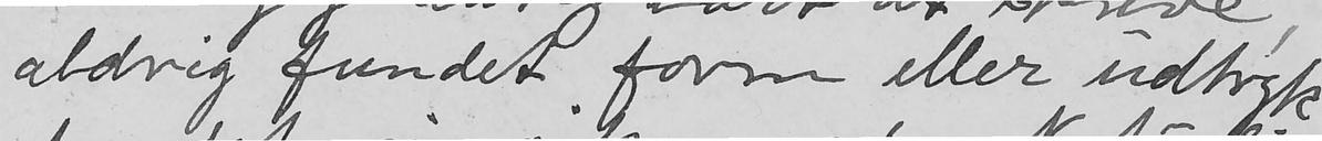aldrig fundet form eller udtryk
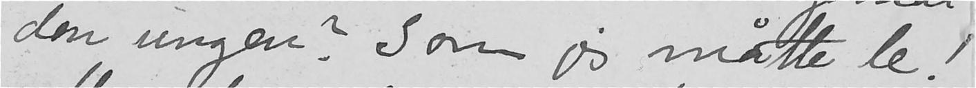den ungen? Som jeg måtte le!
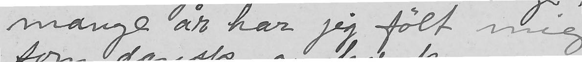mange år har jeg følt mig
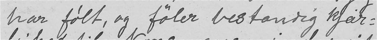har følt, og føler bestandig kjær-
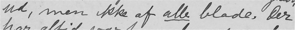ud, men ikke af alle blade. Der
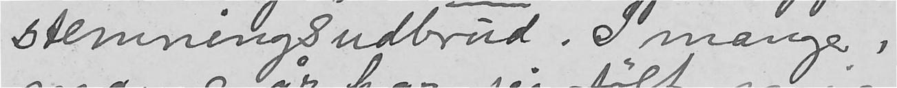stemningsudbrud. I mange,
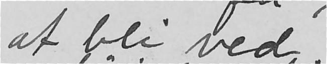at bli ved.
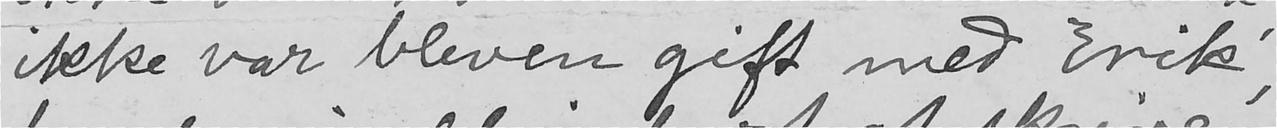ikke var bleven gift med Erik,
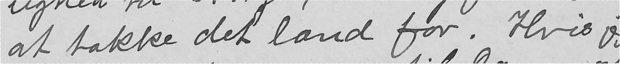at takke det land for. Hvis jeg
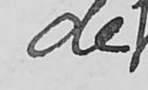de
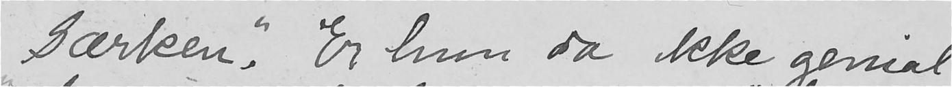"særken". Er hun da ikke genial
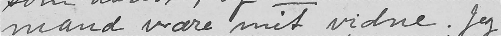mand være mit vidne. Jeg
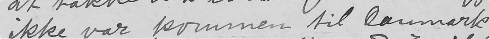ikke var kommen til Danmark,
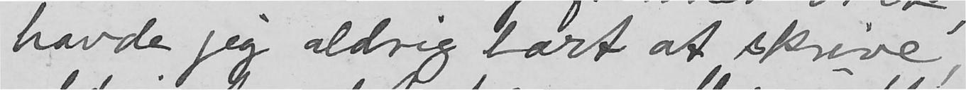havde jeg aldrig lært at skrive,
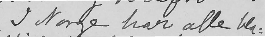I Norge har alle bla-
